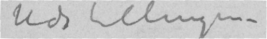Udstilling –
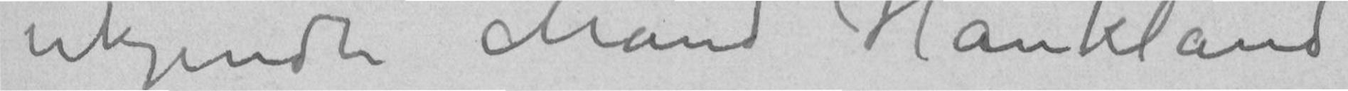ukjendte Mand Haukland
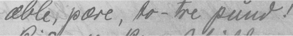æble, pære, to- tre pund!
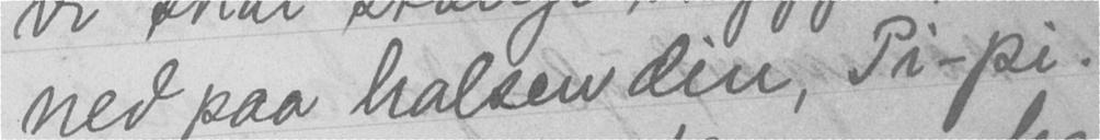ned paa halsen din, Pi-pi.
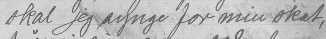skal jeg synge for min skat,
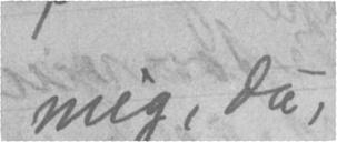mig, da,
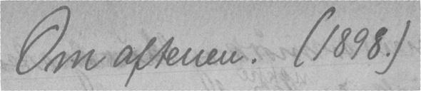Om aftenen. (1898.)
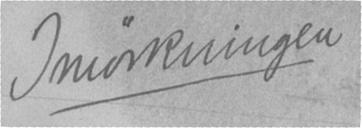I mørkningen
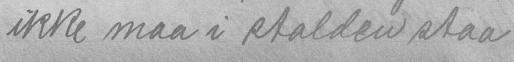ikke maa i stalden staa
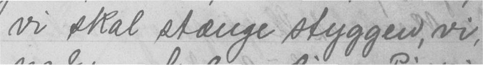vi skal stænge styggen, vi,
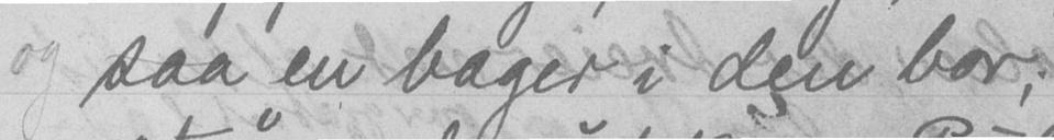saa en bager i den bor;
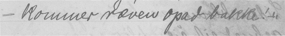- kommer ræven opad bakke! -
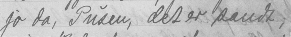jo da, Pusen, det er sandt;
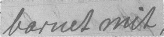barnet mit,
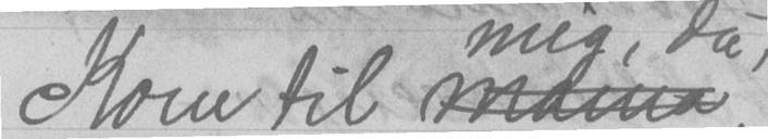Kom til mama,
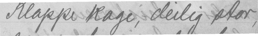Klappe kage, deilig stor,
